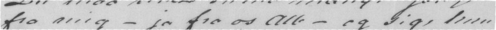fra mig - ja fra os Alle - og sige hun
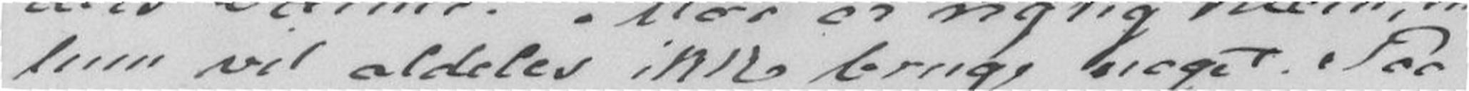hun vil aldeles ikke bruge noget. Paa
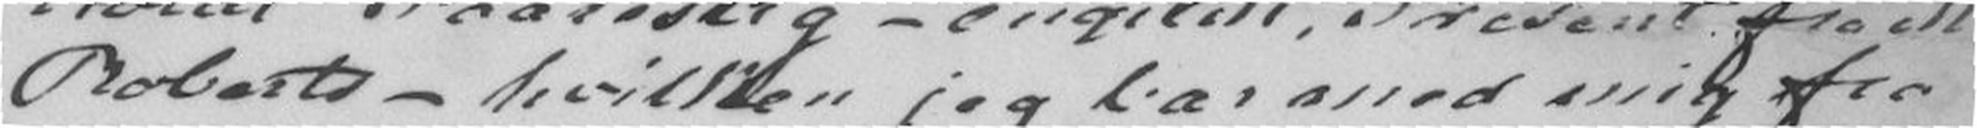Robert - hvilken jeg bar med mig fra
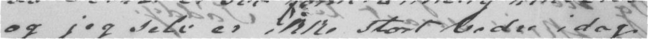og jeg selv er ikke stort bedre idag.
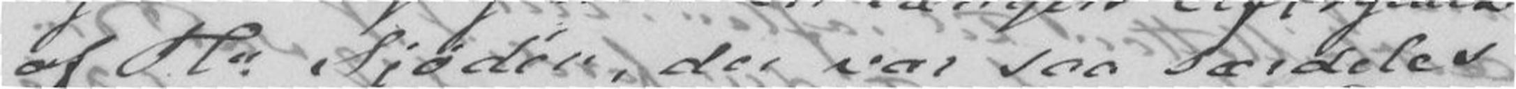af Hr Sjødén, der var saa særdeles
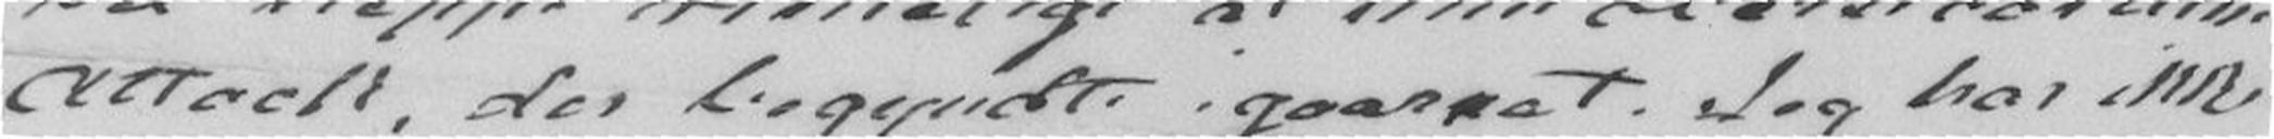Attack, der begyndt igaarnat. Jeg har ikke
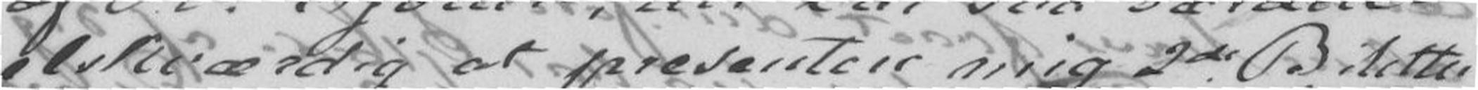elskværdig at presentere mig 2de Biletter
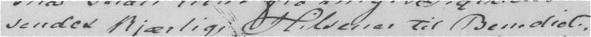sendes kjærlige Hilsener til Benedicte
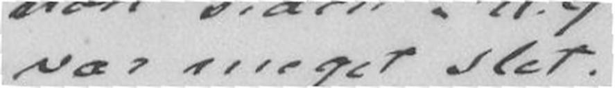var meget slet. -
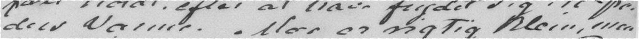ders Varme. Mor er rigtig klein, men
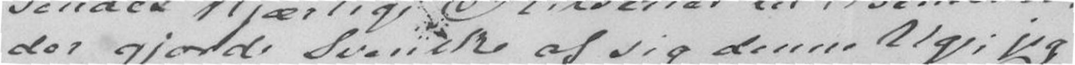der gjorde Svenske af sig denne Uge; jeg
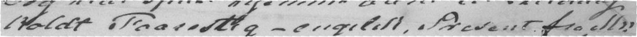koldt Faaresteg - engelsk Present fra Mr
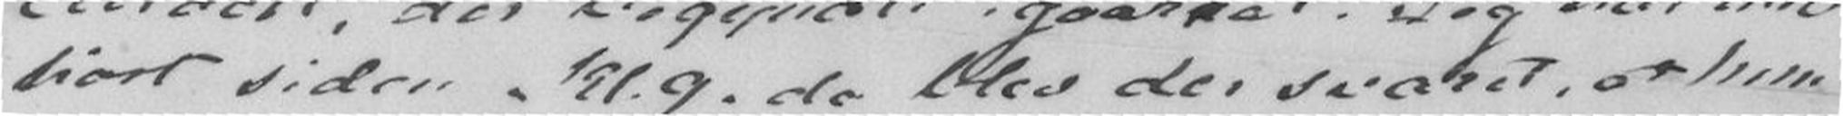hørt siden Kl.9. da blev der svaret, at hun
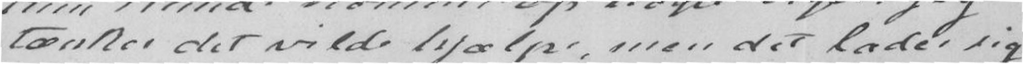tænker det vilde hjælpe, men det lader sig
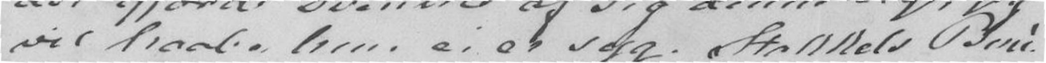vil haabe hun ei er syg Stakkels Bene!
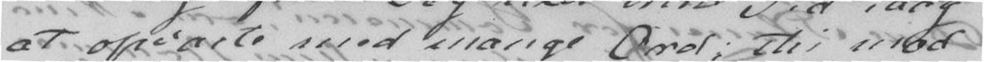at opvarte med Mange Ord; thi mod
